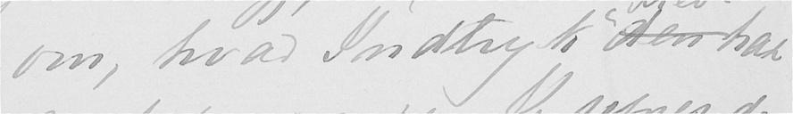om, hvad Indtryk den har
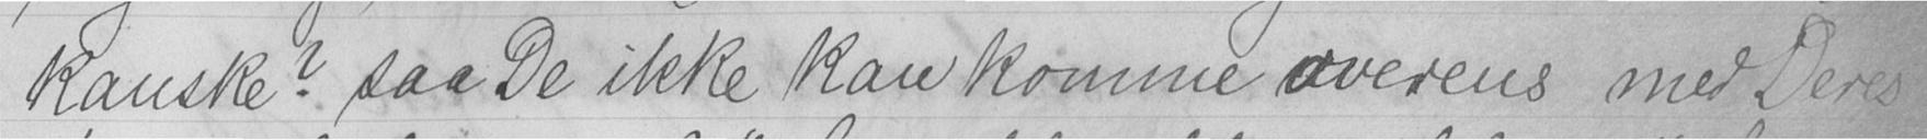kanske? saa De ikke kan komme overens med Deres
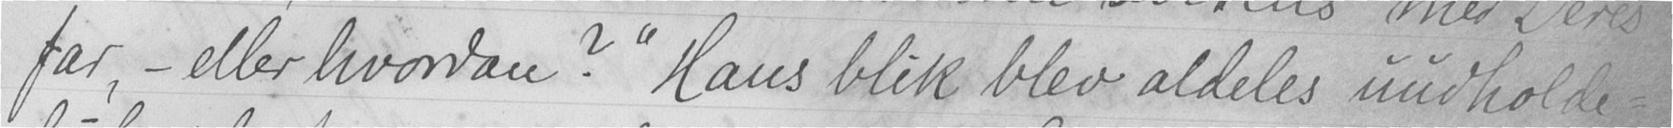far, - eller hvordan?" Hans blik blev aldeles uudholde-
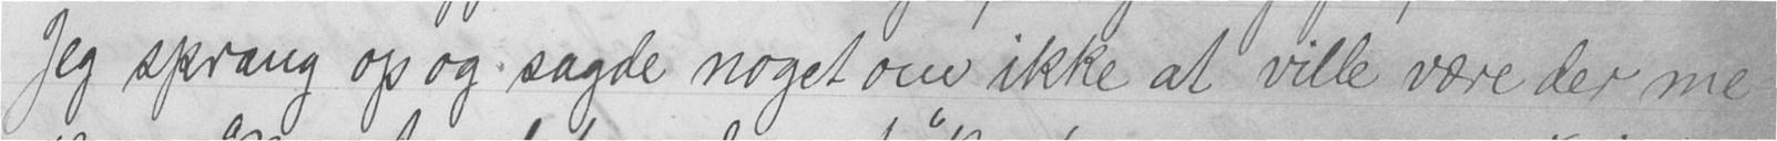Jeg sprang op og sagde noget om ikke at ville være der me-
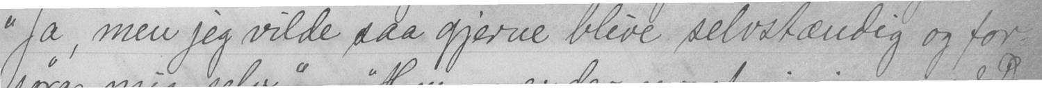"Ja, men jeg vilde saa gjerne blive selvstændig og for-
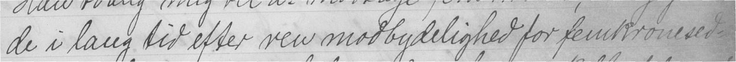de i lang tid efter ren modbydelighed for femkronesed-
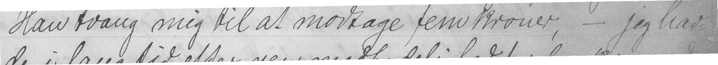Han tvang mig til at modtage fem kroner, - jeg hav-
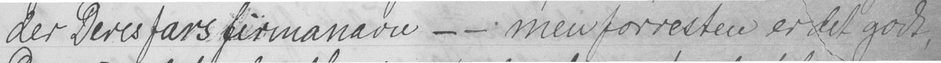der Deres fars firmanavn - - men forresten er det godt,
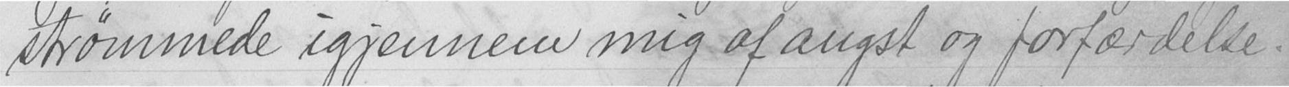strømmede igjennem mig af angst og forfærdelse.
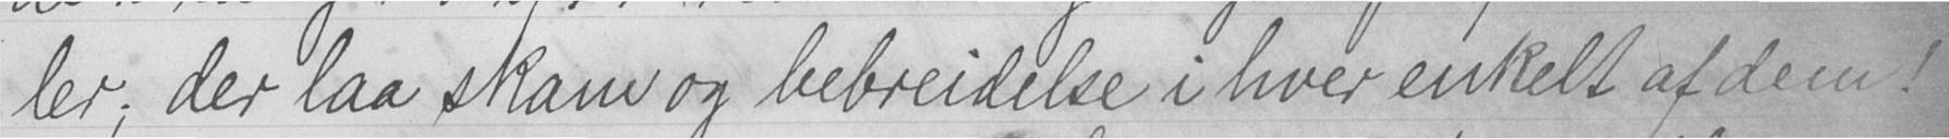ler; der laa skam og bebreidelse i hver enkelt af dem!
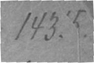143.
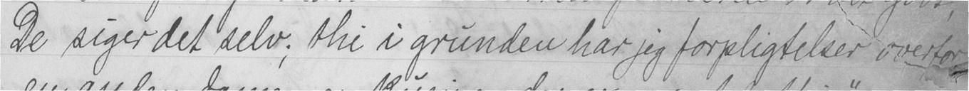De siger det selv; thi i grunden har jeg forpligtelser overfor
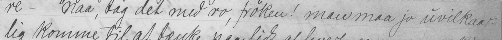re - "naa, tag det med ro, frøken! man maa jo uvilkaar-
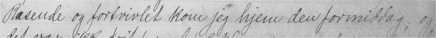Rasende og fortvivlet kom jeg hjem den formiddag; og
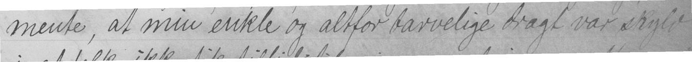mente, at min enkle og altfor tarvelige dragt var skyld
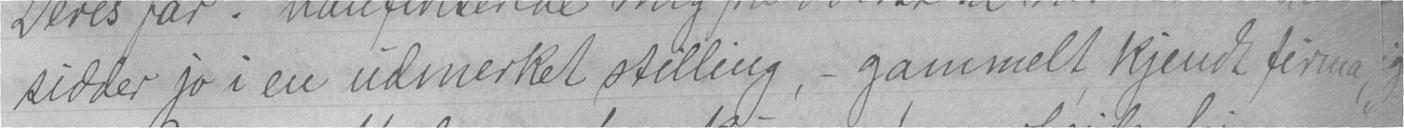sidder jo i en udmerket stilling, - gammelt, kjendt firma,
